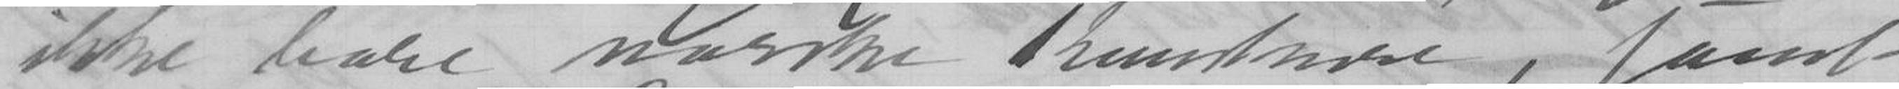ikke bare norske Kunstnere, samt
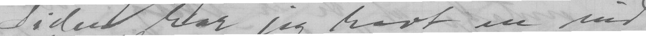Siden har jeg havt en vid-
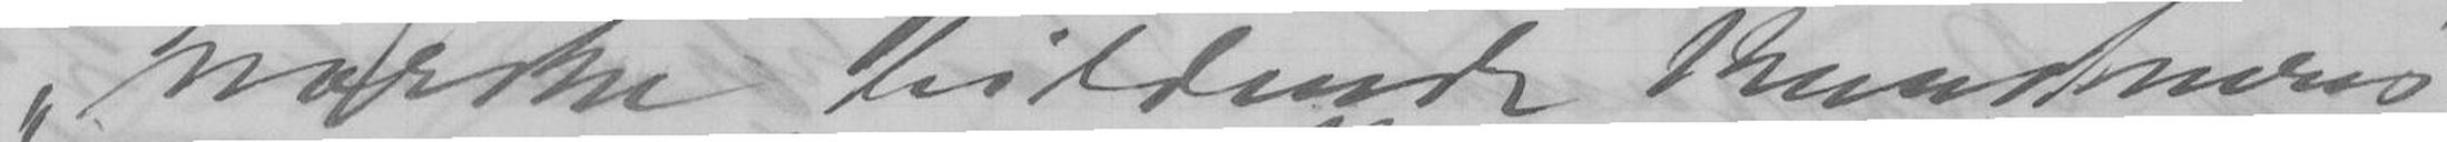norske bildende Kunstneres
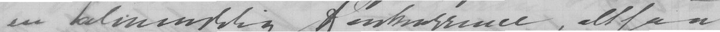en almindelig Konkurrance, altsaa
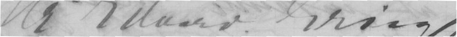Hr. Edvard Grieg
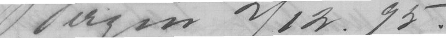Bergen 2/12. 95.
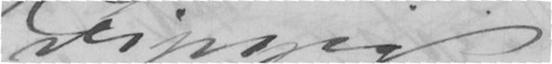Leipzig
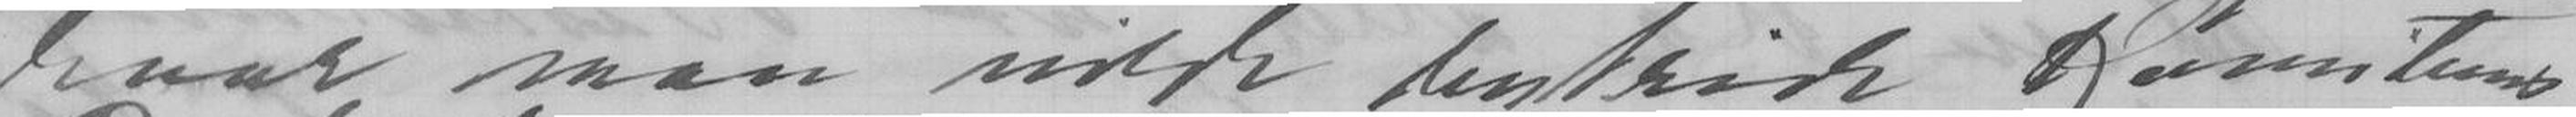hvor man vilde bestride Komiteens
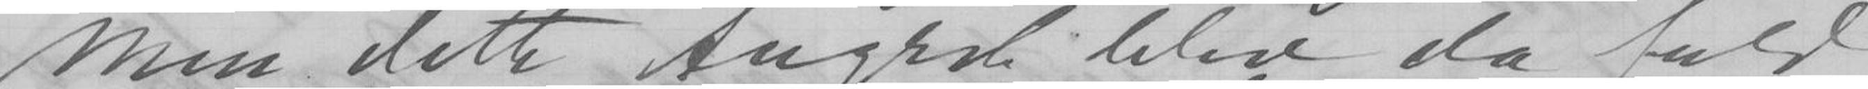Men dette Angreb blev da fuld-
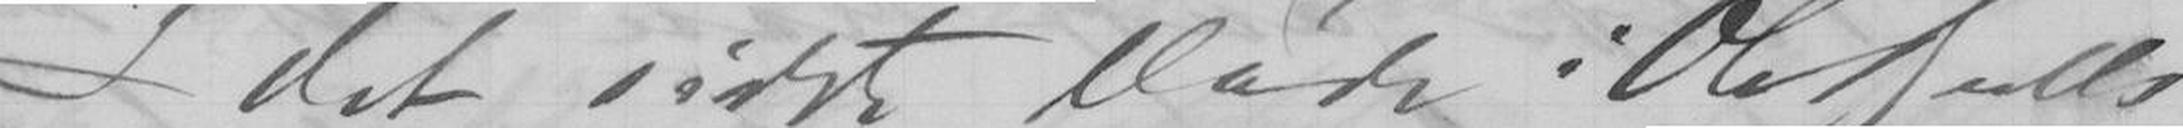I det sidste Møde i Ole Bulls
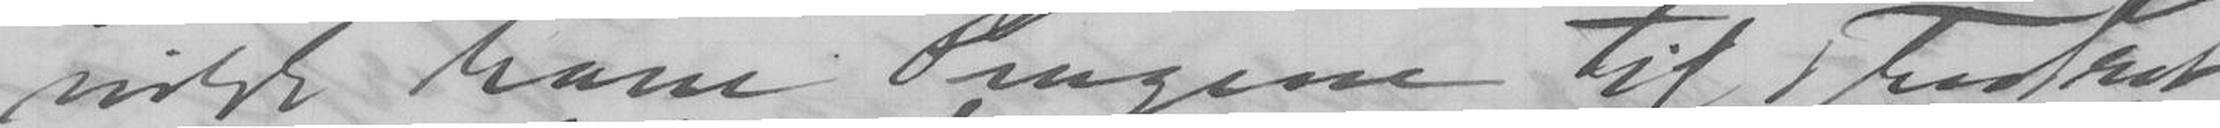vilde have Pengene til Theatret.
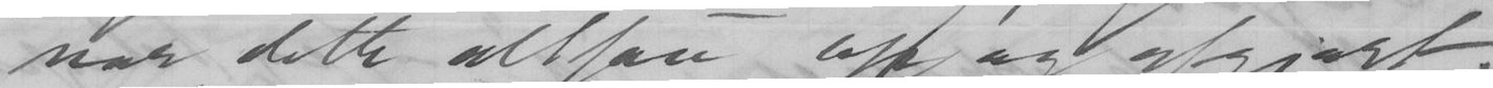var dette altsaa op og afgjort.
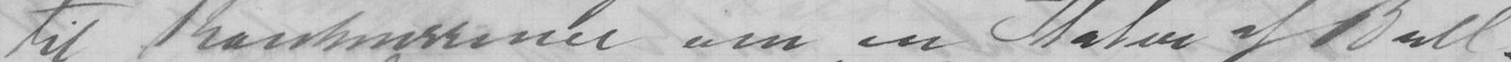til Konkurrance om en Statue af Bull.
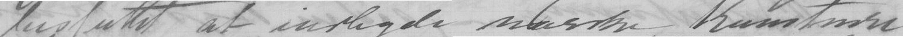besluttet at indbyde norske Kunstnere
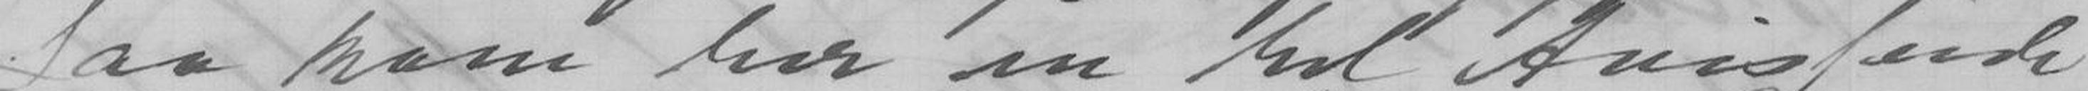Saa kom her en hel Avisfeide
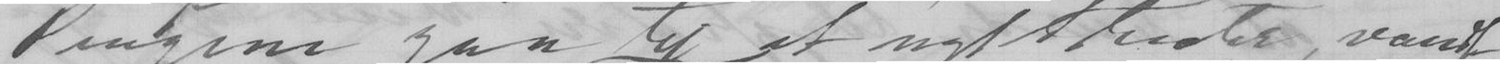Pengene gaa til at nyt theater, vandt
